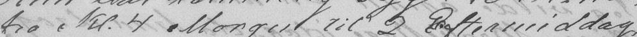fra Kl 4 Morgen til 2 Efter middag
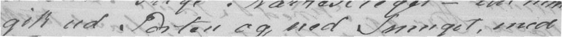gik ud Porten og ned Smuget, med
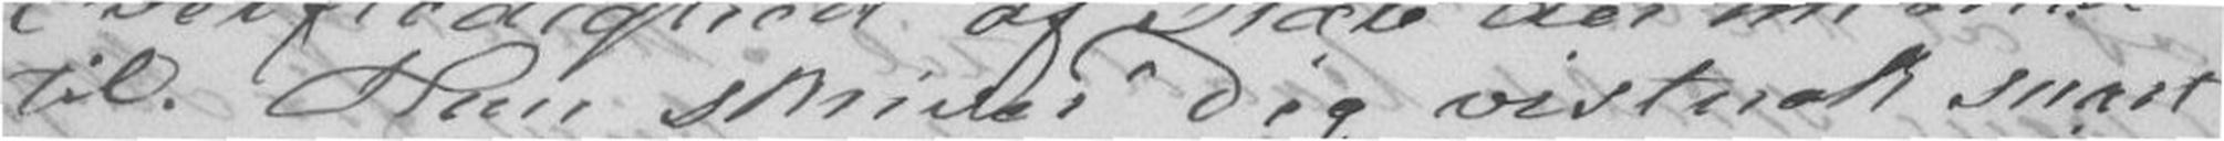til. Hun skriver Dig vistnok snart
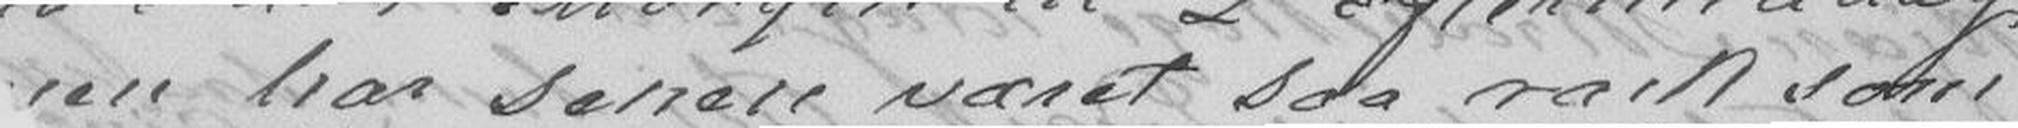en har senere været saa rask som
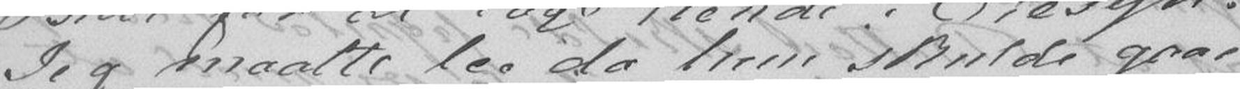Jeg maatte lee da hun skulde gaae
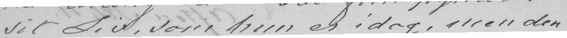sit Liv, som hun er idag, men den
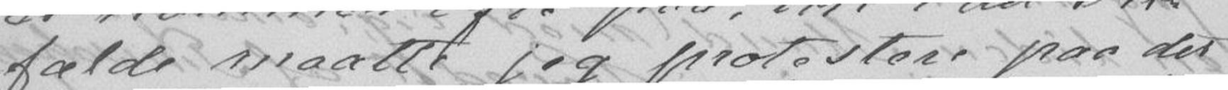fælde maatte jeg protestere paa det
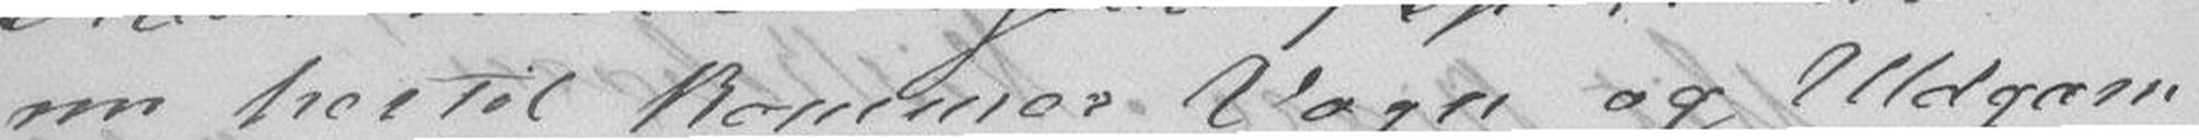nu hertil kommer Vogn og Uldgarn
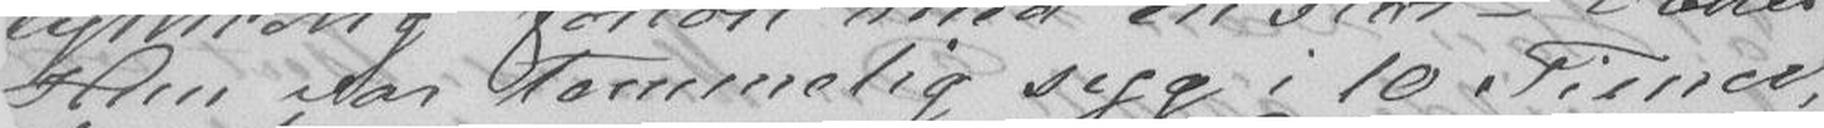Hun var temmelig syg i 10 Timer
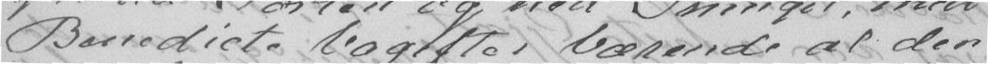Benedicte bagefter bærende al den
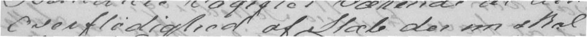overflødighed af Slæb der nu skal
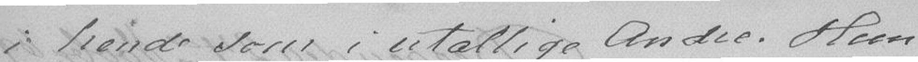i hende som i utallige Andre. Hun
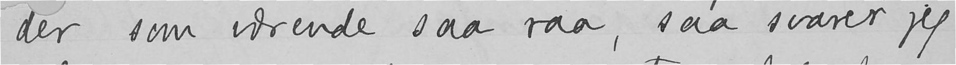der som værende saa raa, saa svarer jeg
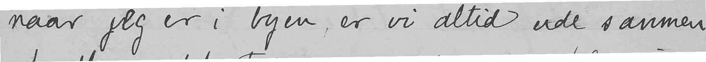naar jeg er i byen, er vi altid ude sammen
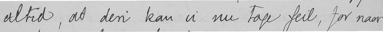altid, at deri kan vi nu tage feil, for naar
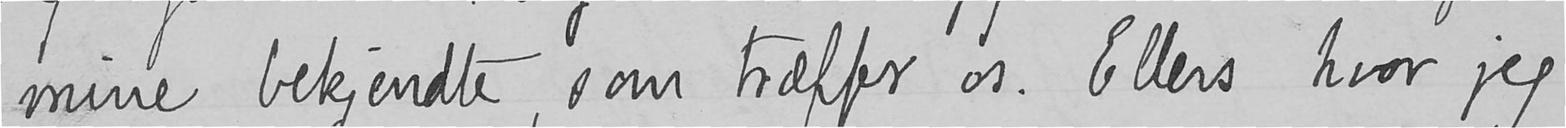mine bekjendte, som træffer os. Ellers hvor jeg
